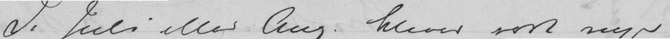I Juli eller Aug. bliver vort nye
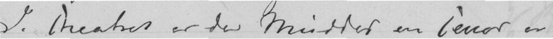I. Theatret er der Meiddes en Tenor er
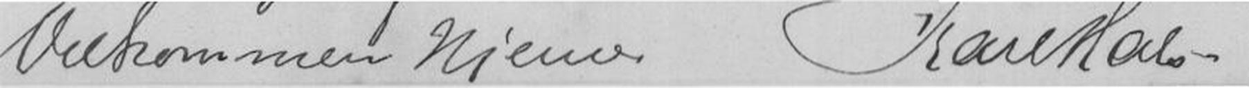Velkommen Hjem. Karl Hals.
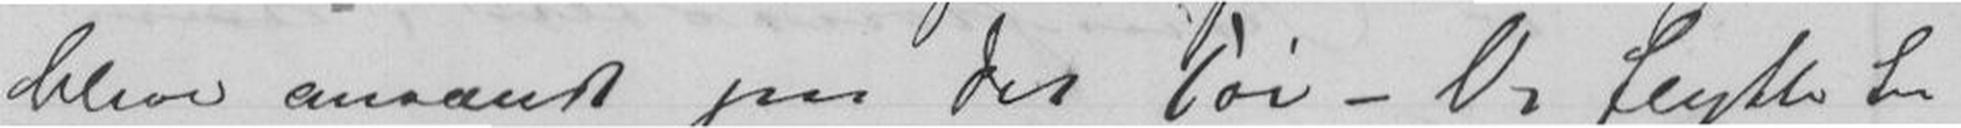blive anvændt paa det Tøv - Vi flytte til
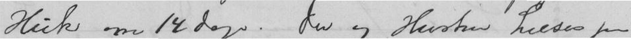Huk om 14 dage. Du og Hustru hilses paa
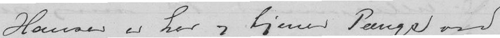Hansen er her og tjener Penge ved
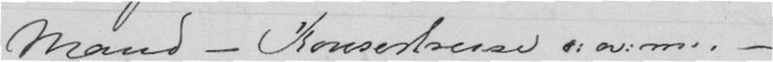Mand - Konsertreise o: ov: m.. -
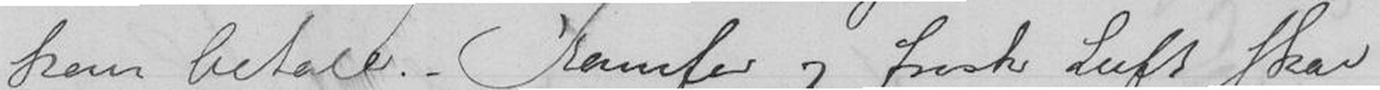kan betale. Kamfer og frisk Luft skal
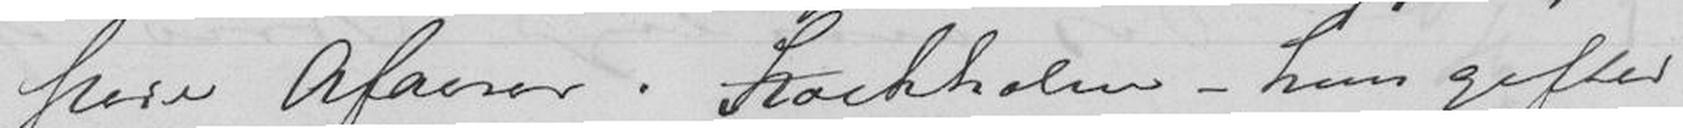store Afaerer. Stockholm - hun gifter
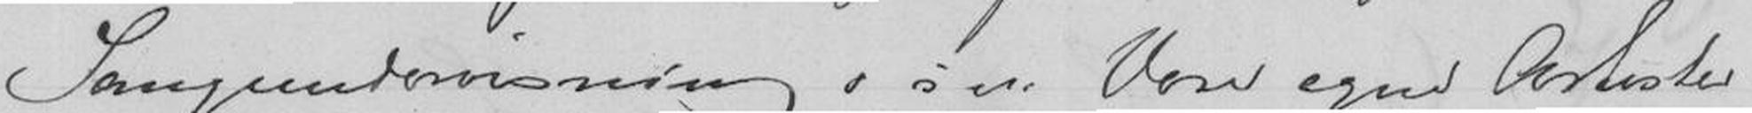Sangundervisning o. s. v. Vore egne Artister
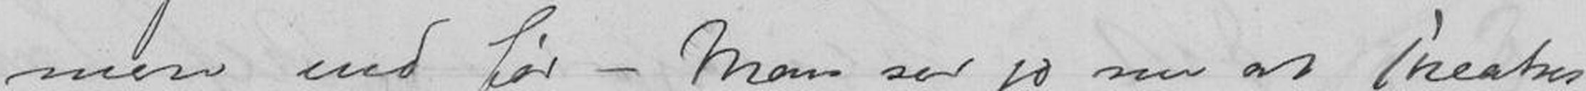mere end før - Man ser jo nu at Theatret
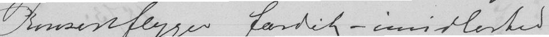Konsertflygel færditg - imidlertid
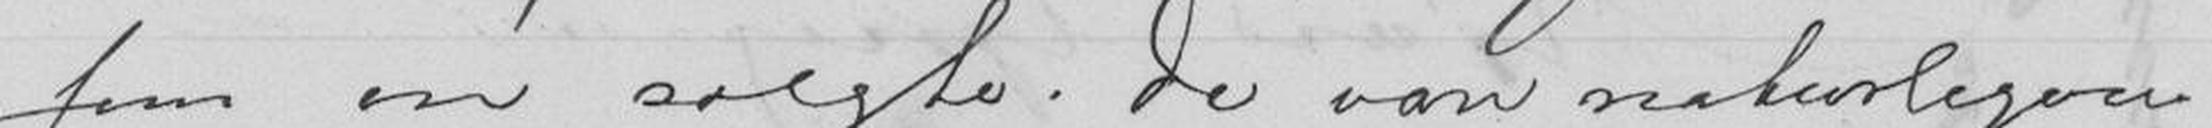som er solgte. De var naturligvis
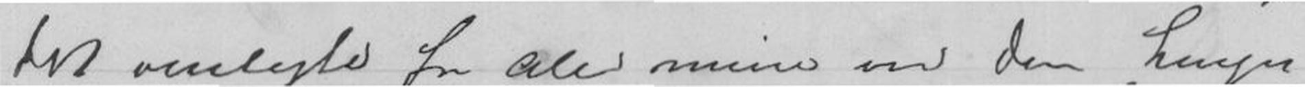det venligte fra Alle mine ved din hengv
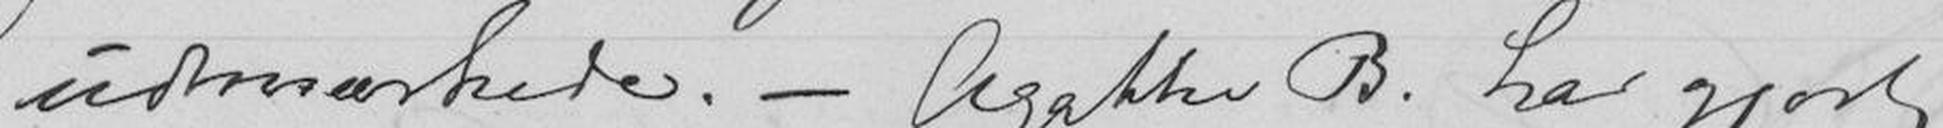udmærkede. - Agathe B. har gjort
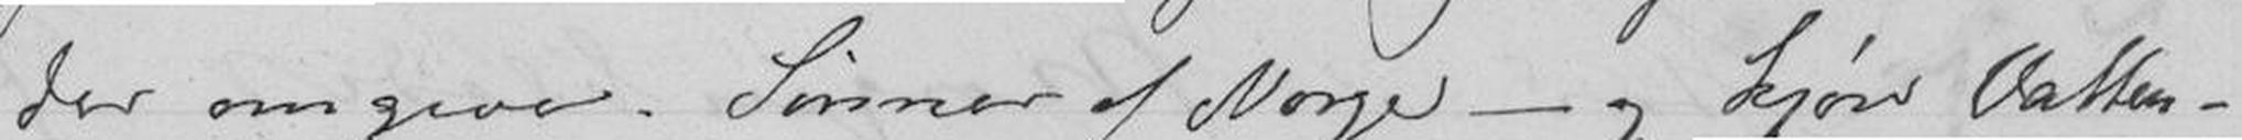der omgive. Sønner af Norge - og Kjøre Vatten-
